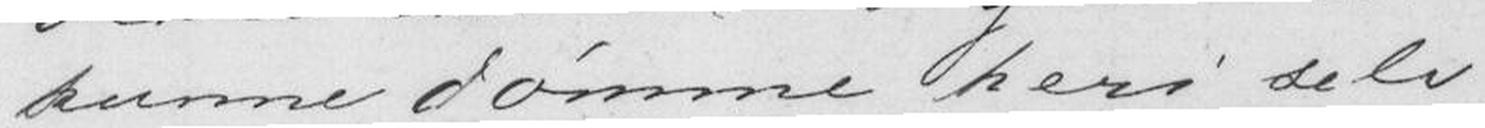kunne dømme heri selv,
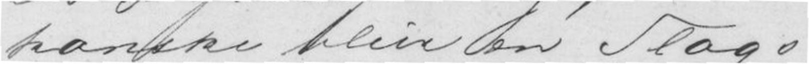kanske blive en Slags
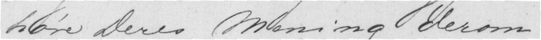høre Deres Mening derom,
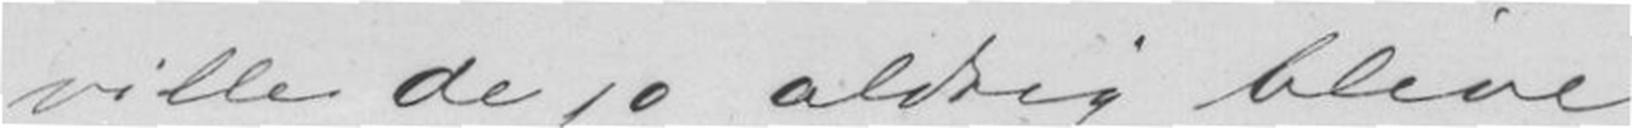ville de jo aldrig blive
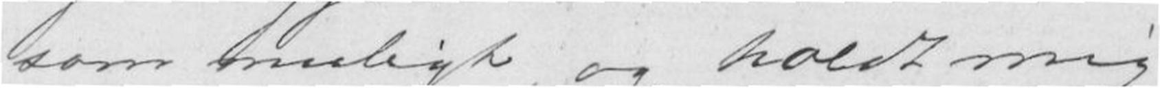som muligt, og holdt mig
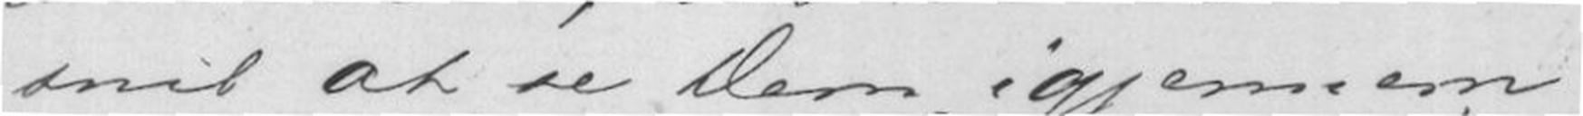snil at se Dem igjennom
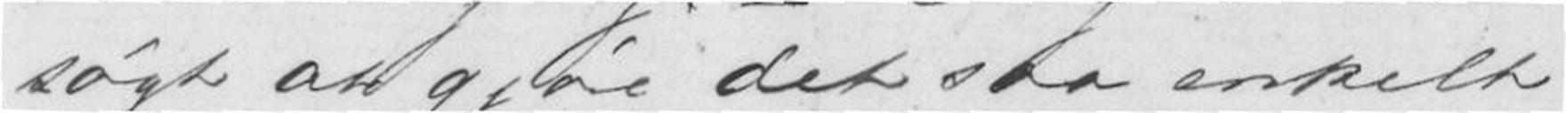søgt at gjøre det saa enkelt
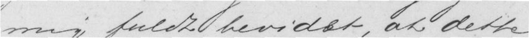mig fuldt bevidst, at dette
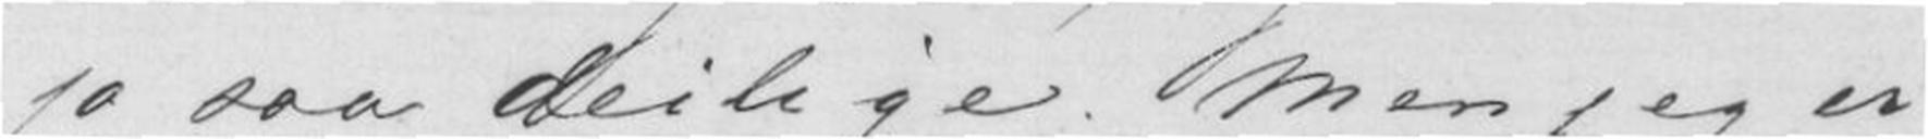jo saa deilige. Men jeg er
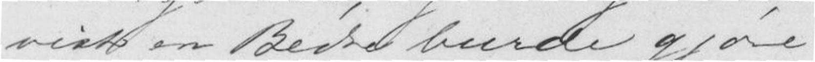vist en Bedre burde gjøre
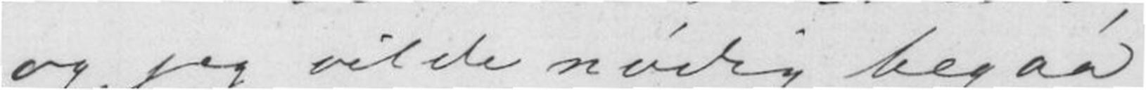og jeg vilde nødig begaa
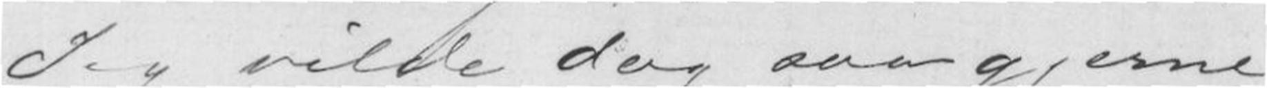Jeg vilde dog saa gjerne
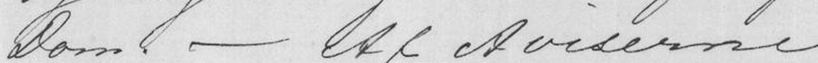Dom.- Af Aviserne
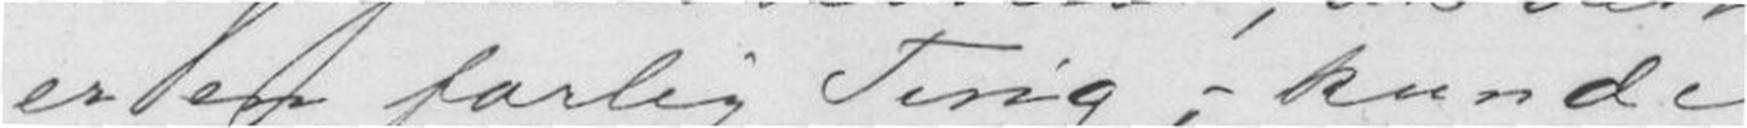er en farlig Ting; kunde
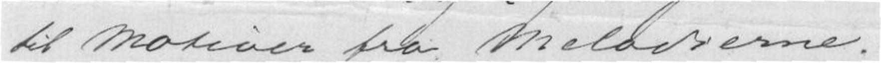til Motiver fra Melodierne.-
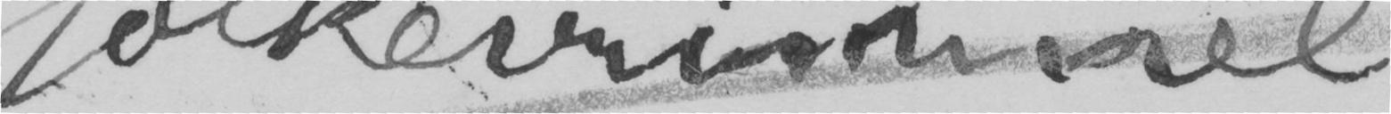folkevrimmel.
